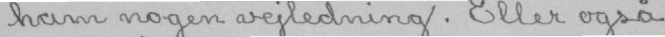ham nogen vejledning. Eller også
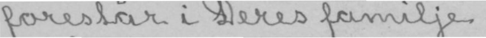forestår i Deres familje.
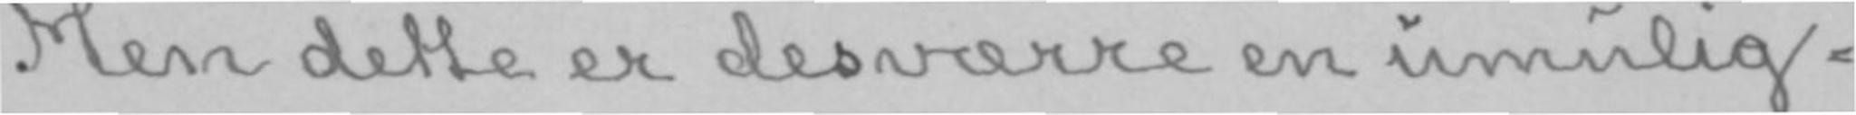Men dette er desværre en umulig-
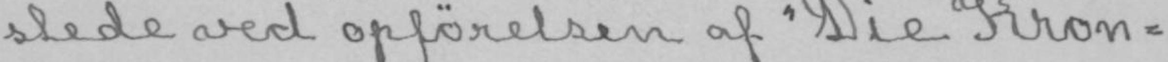stede ved opførelsen af «Die Kron-
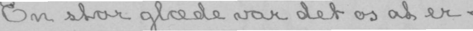En stor glæde var det os at er-
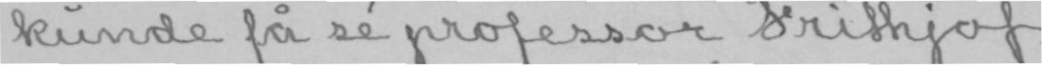kunde få sé professor Frithjof
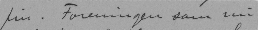fin. Foreningen som nu
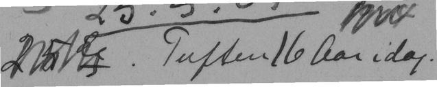. Tuften 16 Aar idag.
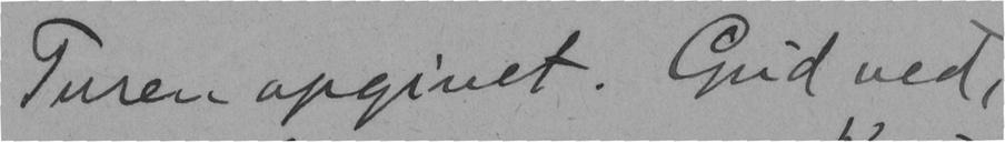Turen opgivet. Gud ved,
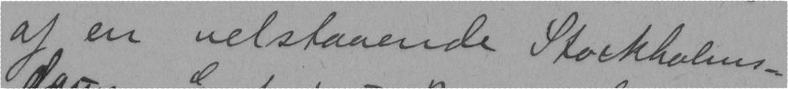af en velstaaende Stockholms-
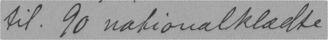til. 90 nationalklædte
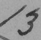13
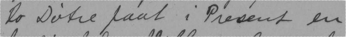to Døtre faat i Present en
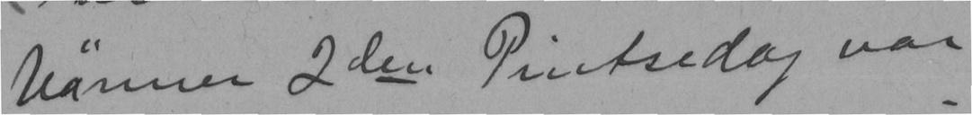vänner 2den Pintsedag var
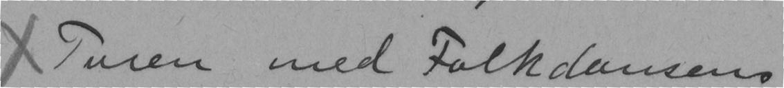x Turen med Folkdansens
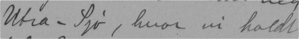Utra - Sjø, hvor vi holdt
